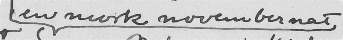en mork novembernat
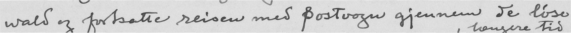wald og fortsatte reisen med postvogn gjennem de løse
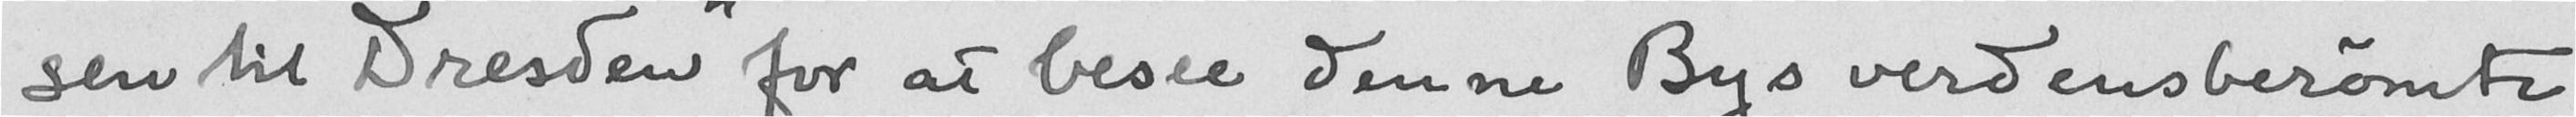sen til Dresden "for at besee denne Bys verdensberømte
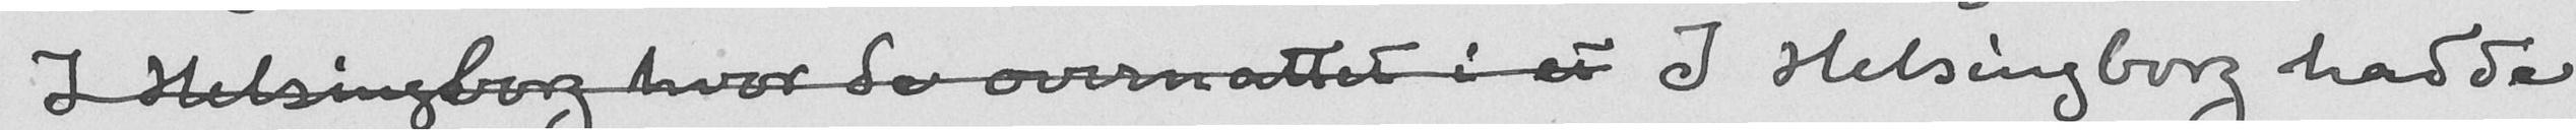I Helsingborg hvor de overnattet i et I Helsingborg hadde
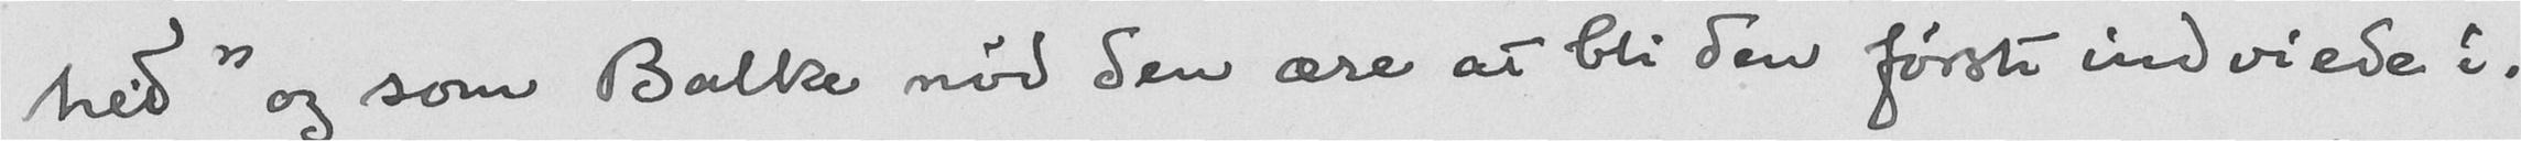hed" og som Balke nød den ære at bli den første indviede i.
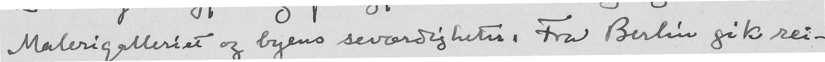Malerigalleriet og byens seværdigheter. Fra Berlin gik rei-
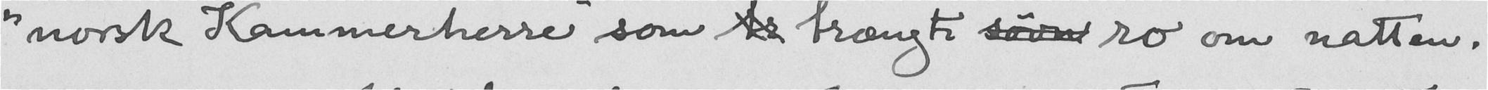"norsk Kammerherre" som tr trængte søvn ro om natten.
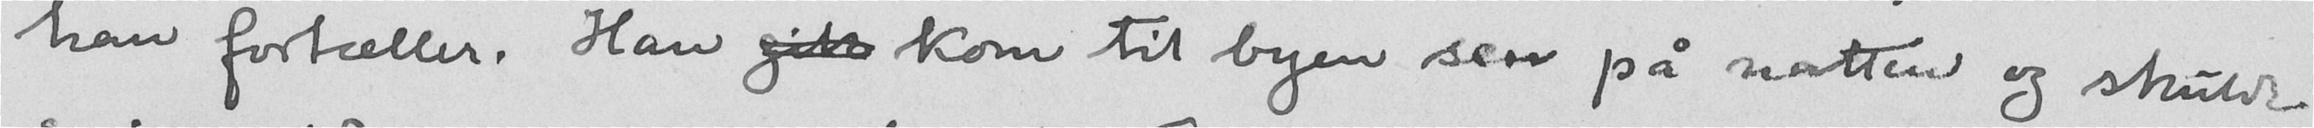han fortæller. Han gik kom til byen sen på natten og skulde
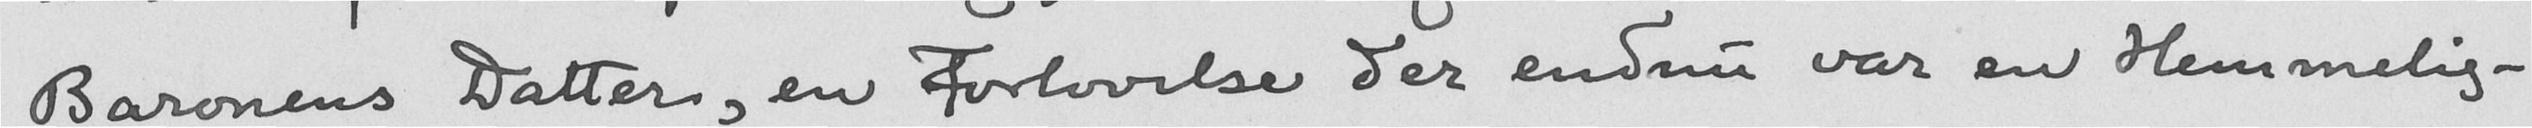Baronens Datter, en Forlovelse der endnu var en Hemmelig-
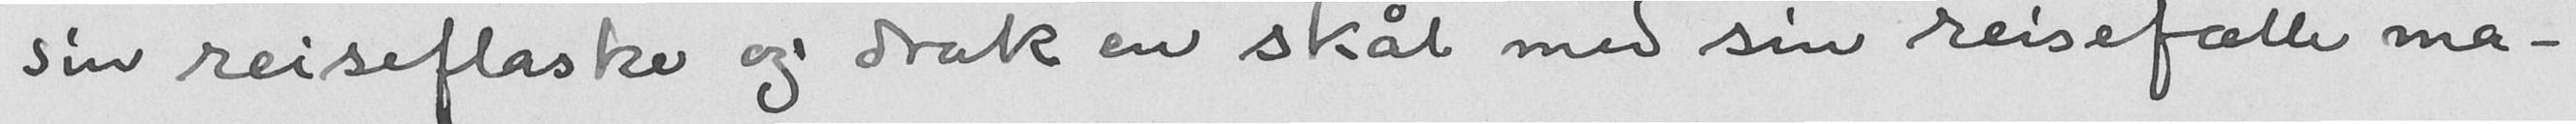sin reiseflaske og drak en skål med sin reisefælle ma-
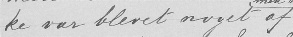ke var blevet noget af
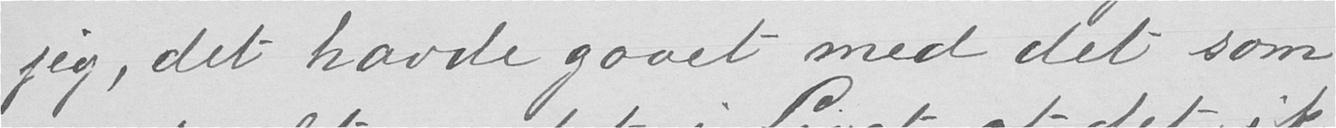jeg, det havde gaaet med det som
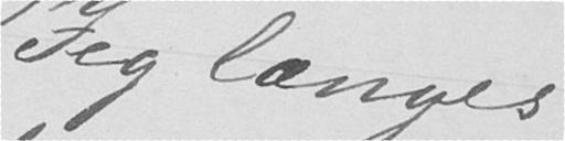Jeg længes
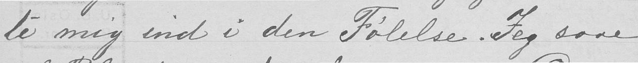te mig ind i den Følelse. Jeg saae
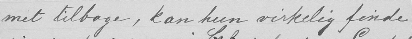met tilbage, kan hun virkelig finde
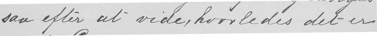saa efter at vide, hvorledes det er
